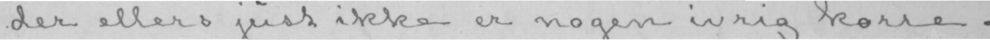der ellers just ikke er nogen ivrig korre-
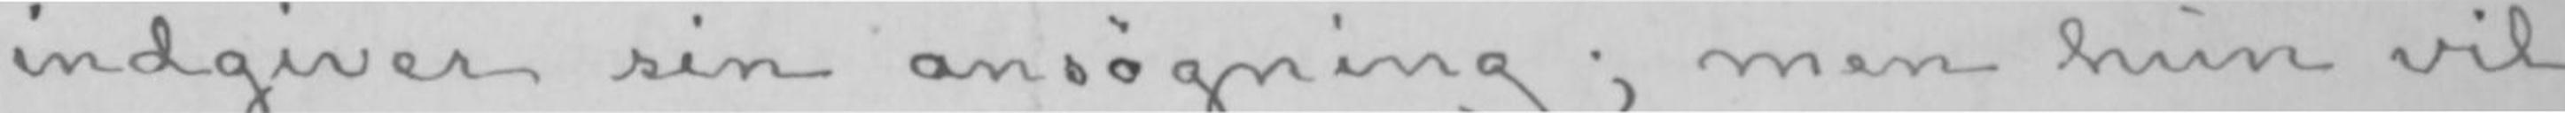indgiver sin ansøgning; men hun vil
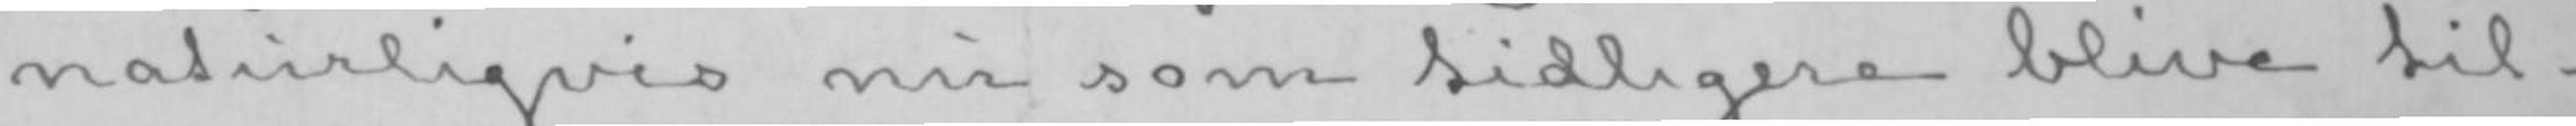naturligvis nu som tidligere blive til-
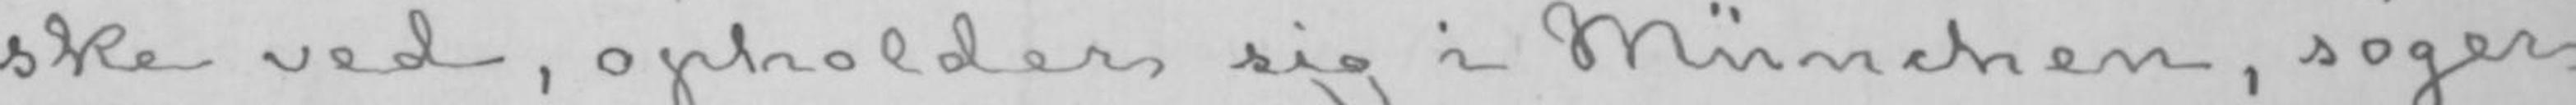ske ved, opholder sig i München, søger
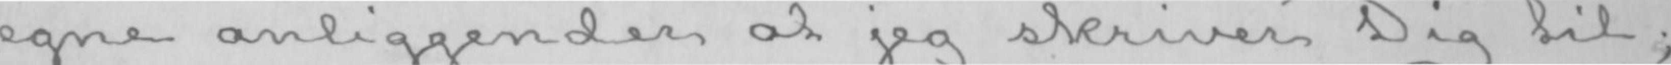egne anliggender at jeg skriver Dig til;
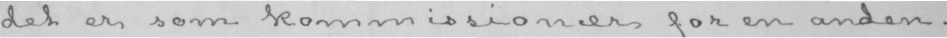det er som kommissionær for en anden.
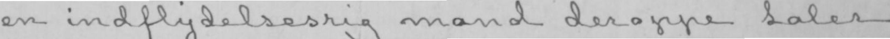en indflydelsesrig mand deroppe taler
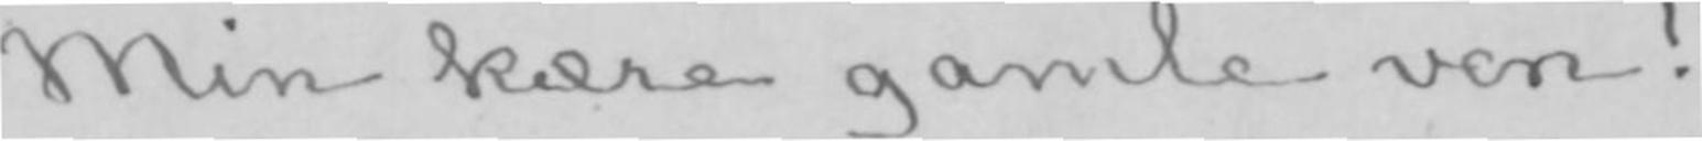Min kære gamle ven!
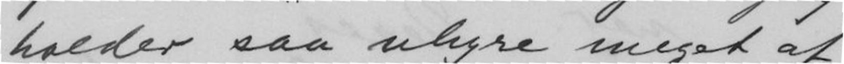holder saa uhyre meget af
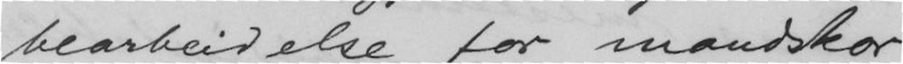bearbeidelse for mandskor
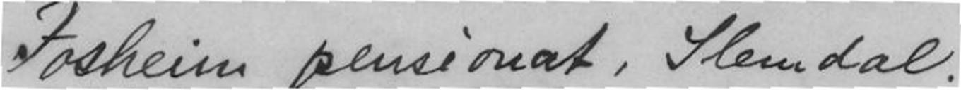Fosheim pensionat, Slemdal.
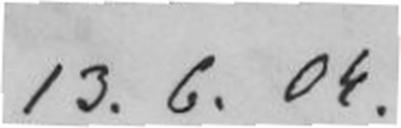13.6.04.
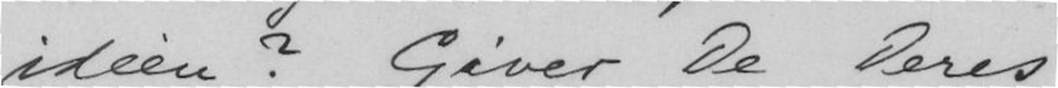ideen? Giver De Deres
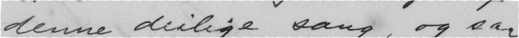denne deilige sang, og saa
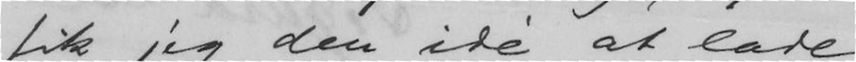fik jeg den ide at lade
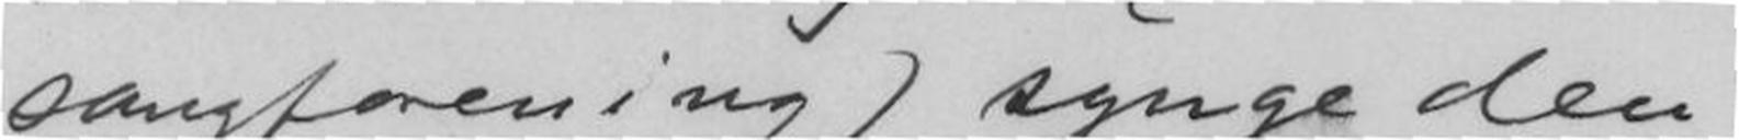sangforening) synge den.
